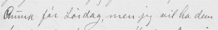Ruma før Lørdag, men jeg vil ha dem
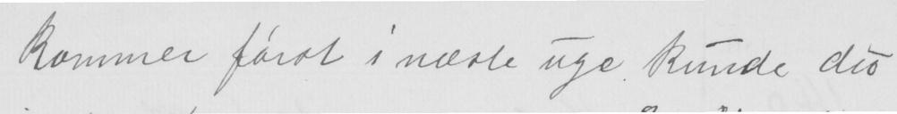kommer først i næste uge. Kunde du
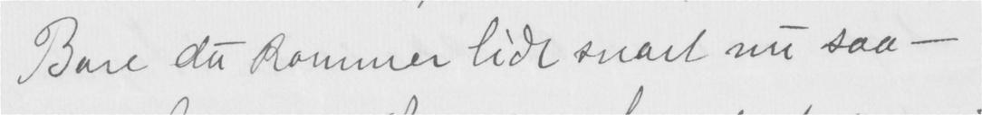Bare du kommer lidt snart nu saa –
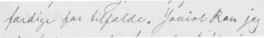færdige for tilfælde. Javist kan jeg
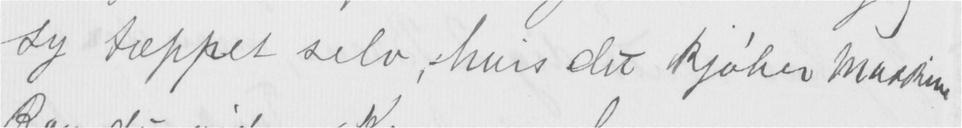sy tæppet selv, hvis du kjøber maskin
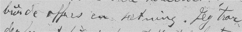burde offres en sætning. Jeg tror
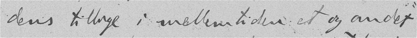dens tillige i mellemtiden et og andet
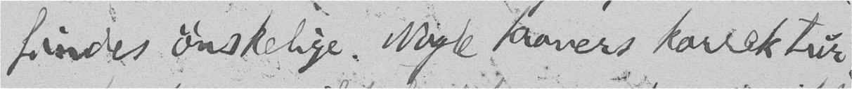findes ønskelige. Nogle kroners korrektur-
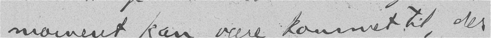moment kan være kommet til, der
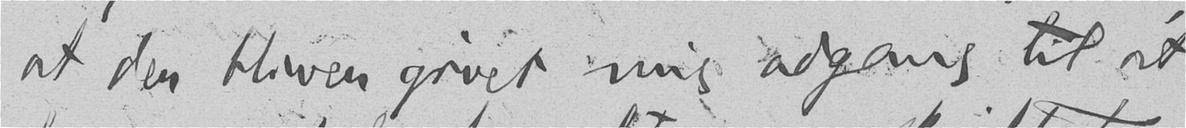at der bliver givet mig adgang til at
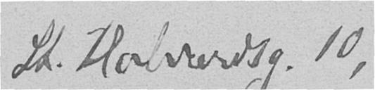St. Halvardsg. 10,
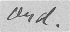ord.
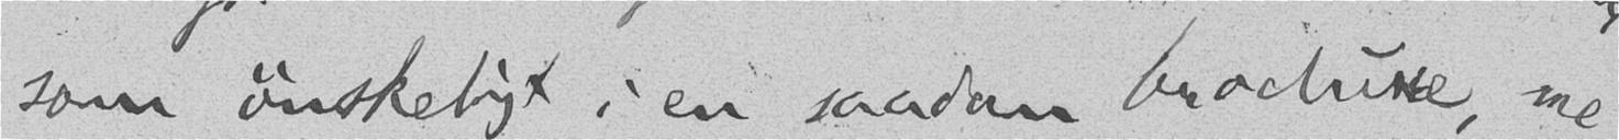som ønskeligt i en saadan brochure, me-
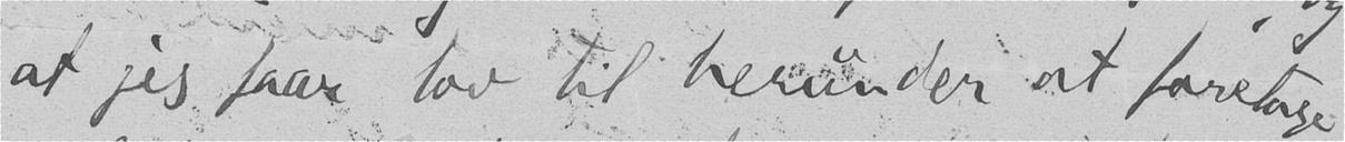at jeg faar lov til herunder at foretage
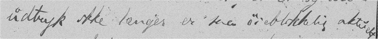udtryk ikke længer er saa øieblikkelig aktuelt
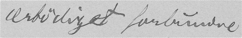ærbødigst forbundne
